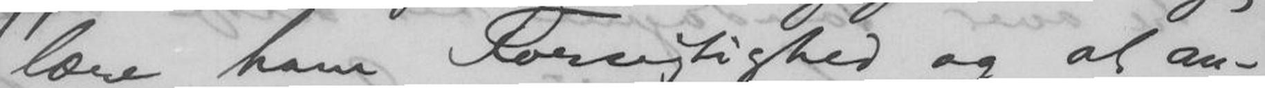lære ham Forsigtighed og at an-
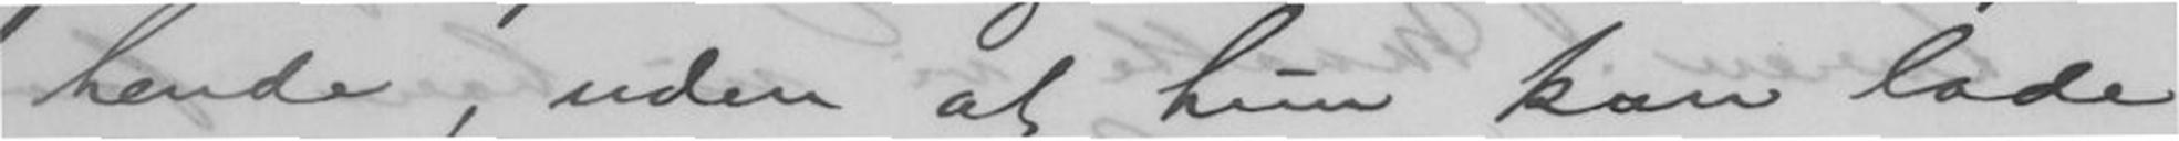hende, uden at hun kan lade
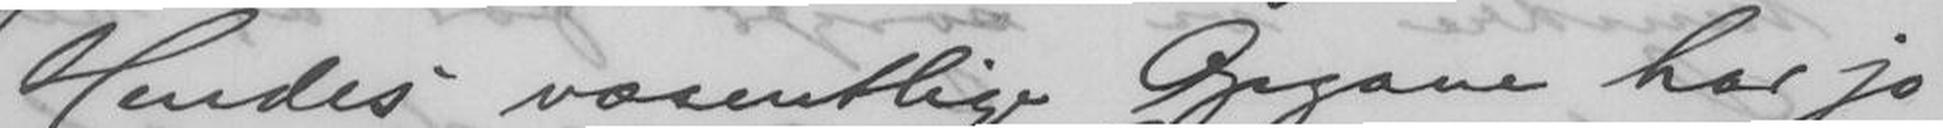Hendes væsentlige Opgave har jo
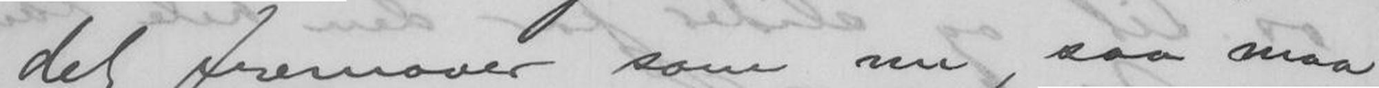det fremover som nu, saa maa
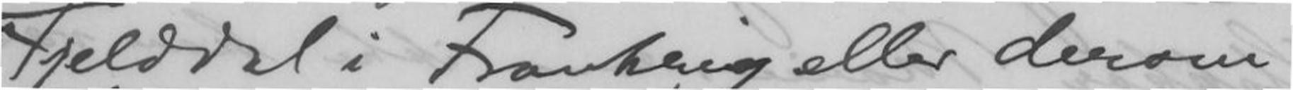Fjelddal i Frankrig eller derom-
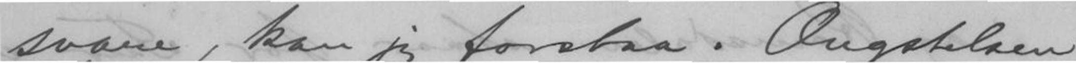svare, kan jeg forstaa. Ængstelsen
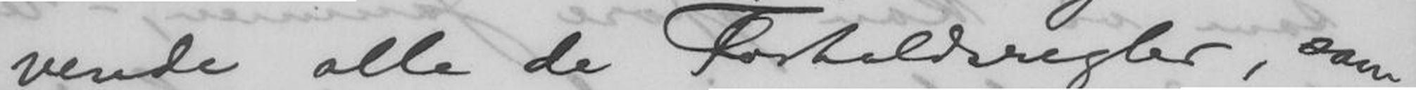vende alle de Forholdsregler, som
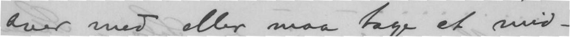over med eller maa tage et mid-
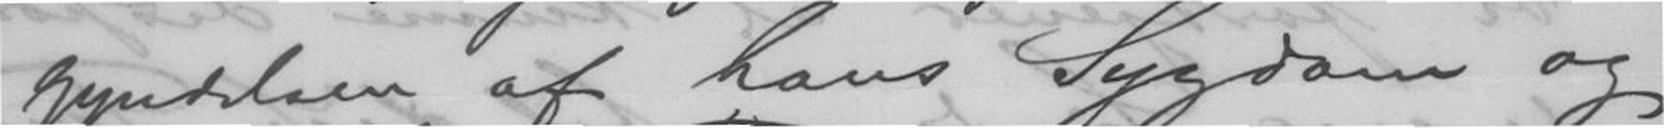gyndelsen af hans Sygdom og
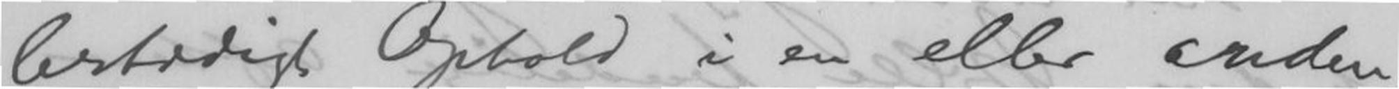lertidigt Ophold i en eller anden
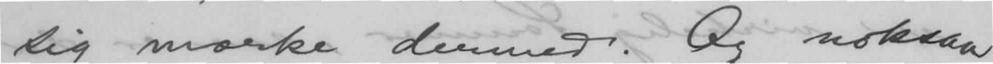sig mærke dermed. Og noksaa
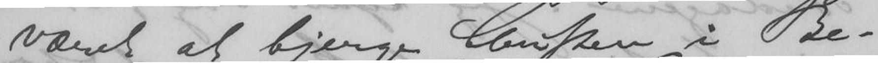været at bjerge Christen i Be-
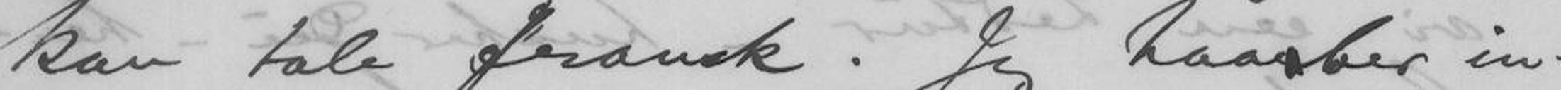kan tale fransk. Jeg haaber in-
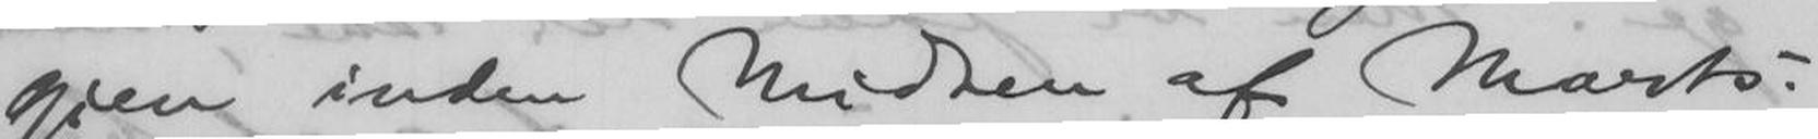gjen inden Midten af Marts.
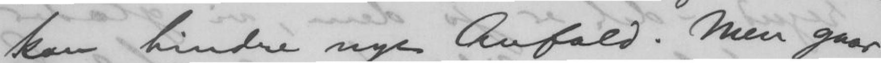kan hindre nye Anfald. Men gaar
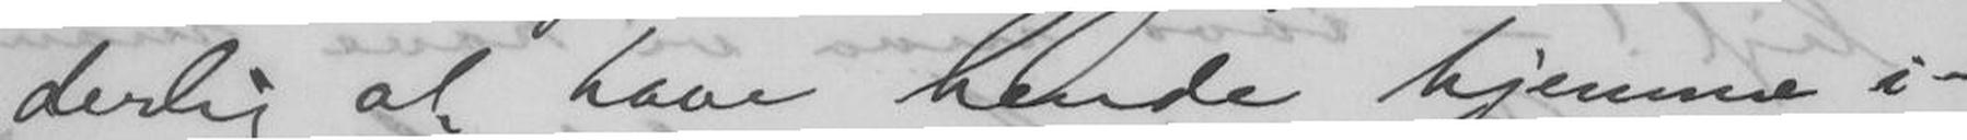derlig at have hende hjemme i-
